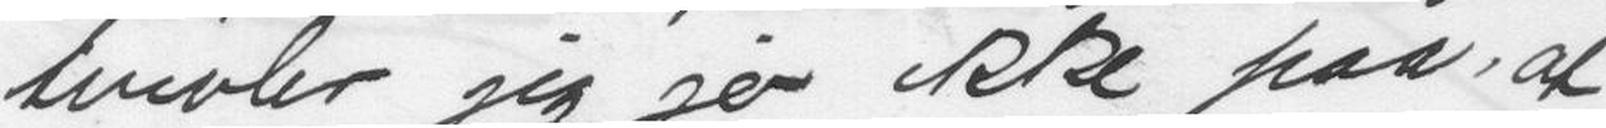tvivler jeg jo ikke paa, at
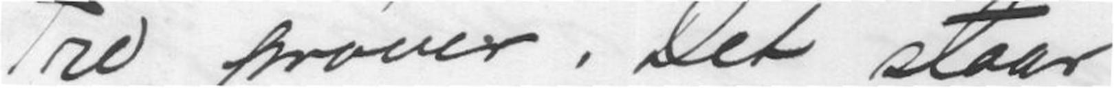Tre prøver. Det staar
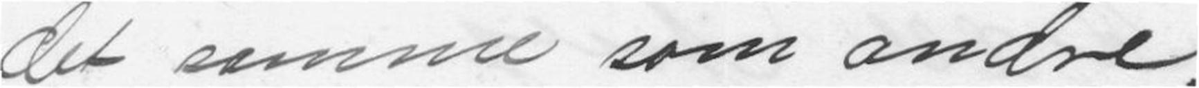det samme som andre.
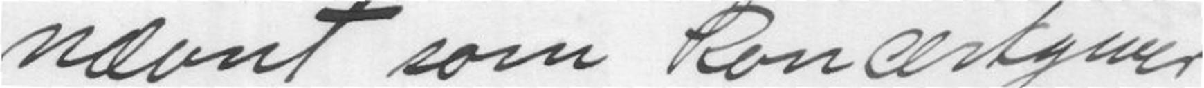nævnt som koncertgiver,
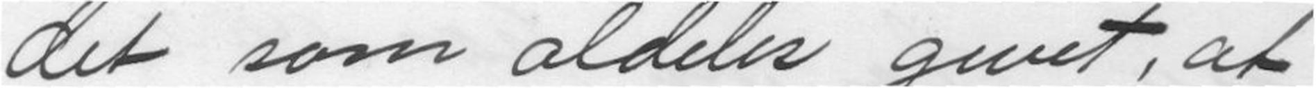det som sldeles givet, at
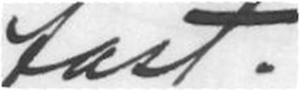fast.
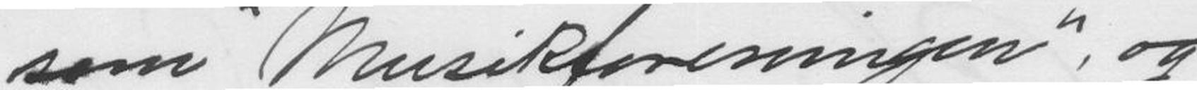som "Musikforeningen", og
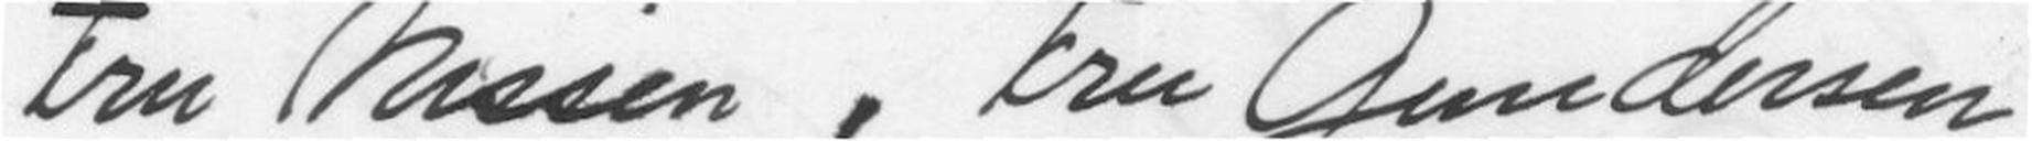Fru Nissen, Fru Gundersen
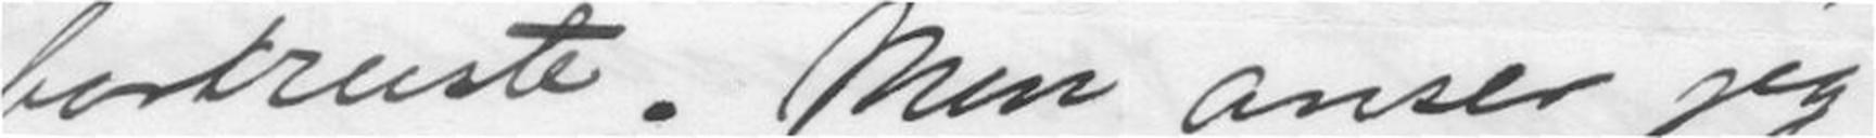bortreiste. Men anser jeg
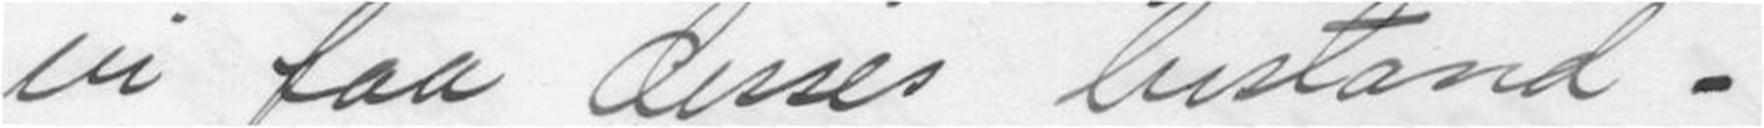vi faa disses bistand -
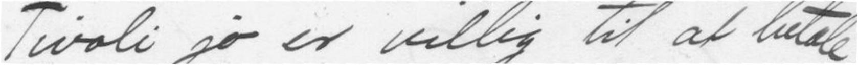Tivoli jo er villig til at betale
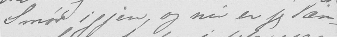Smør igjen, og nu er jeg læn-
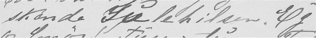skende Julehilsen. Eg
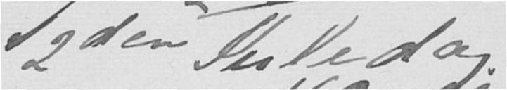2den Juledag.
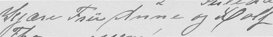Kjære Fru Anne og Rolf
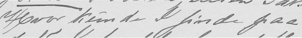Hvor kunde I finde paa
